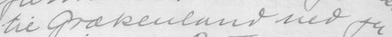til Grækenland ved jeg
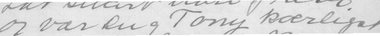og vær Du og Tony kærligst
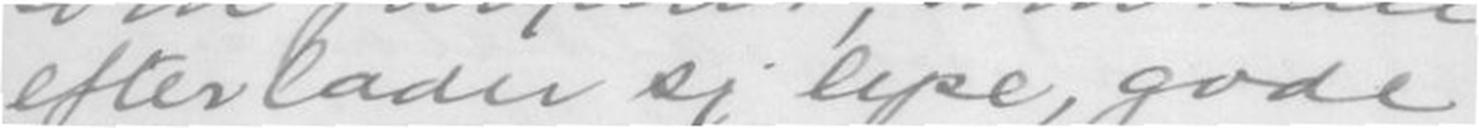efterlader sig lyse, gode
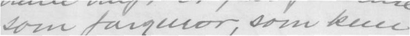som far og mor, som kun
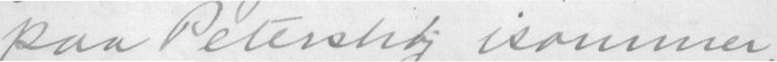paa Petershøj isommer.
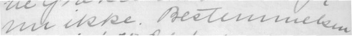nu ikke Bestemmelsen
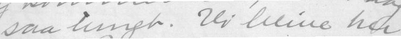saa tungt. Vi blive her
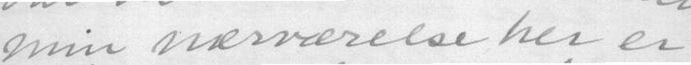min nærværelse her er
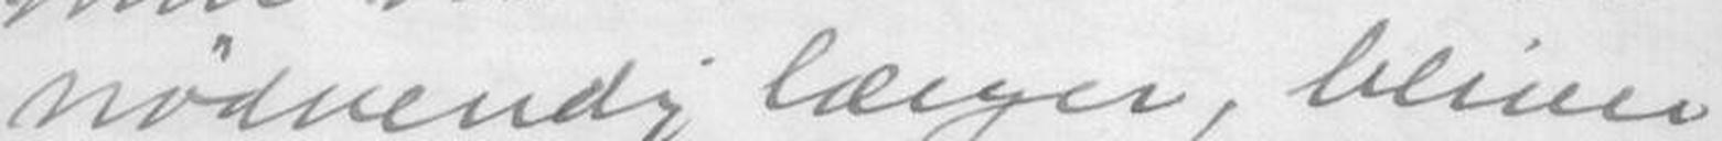nødvendig længer, bliver
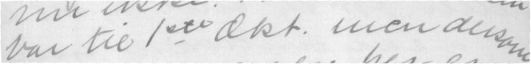var til 1ste Okt. men desverre
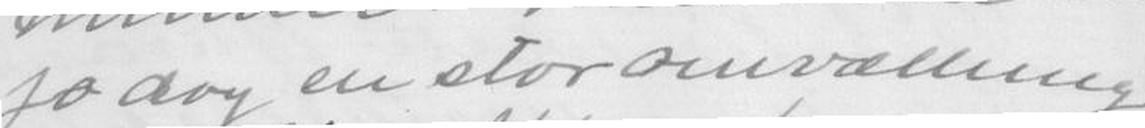jo dog en stor omvæltning
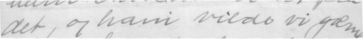det, og ham vilde vi gærne
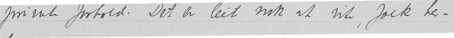private forhold. Det er leit nok at vite, folk her-
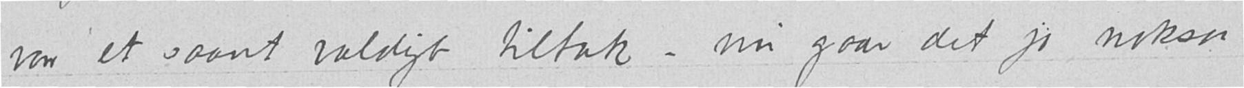var et saant vældigt tiltak — nu gaar det jo noksaa
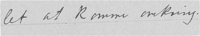let at komme omkring.
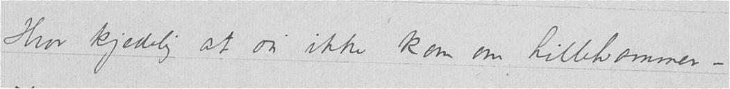Hvor kjedelig at du ikke kom om Lillehammer —
